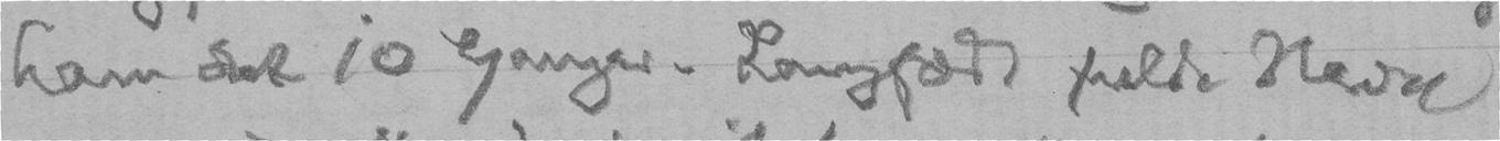ham det 10 Ganger. Langfeldt fulde Navn
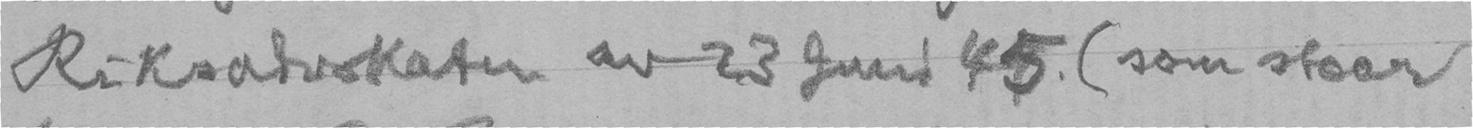Riksadvokaten av 23 Juni 45 (som staar
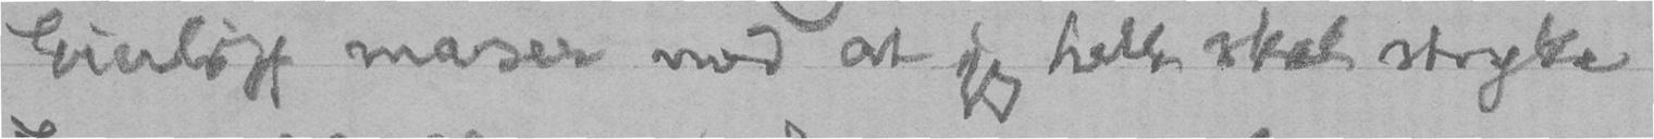Gierløff maser med at jeg helt skal stryke
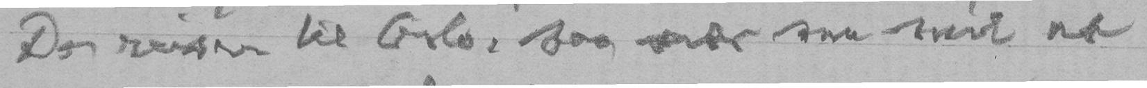De reiser til Oslo, saa vær saa snil at
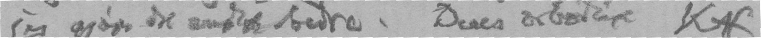jeg gjøre det endda bedre. Deres ærbødige KH
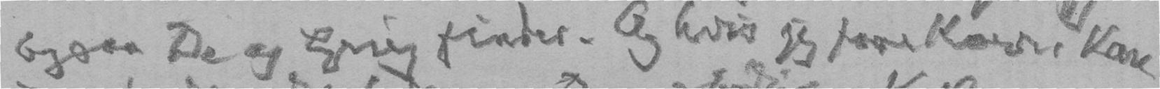ogsaa De og Grieg finder. Og hvis jeg faar Korr, kan
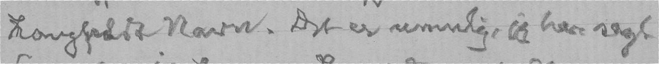Langfeldt Navn. Det er umulig, jeg har sagt
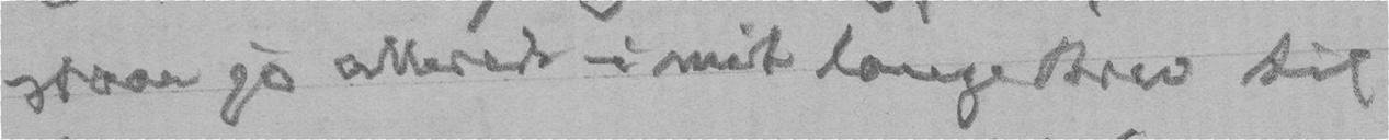staar jo allerede i mit lange Brev til
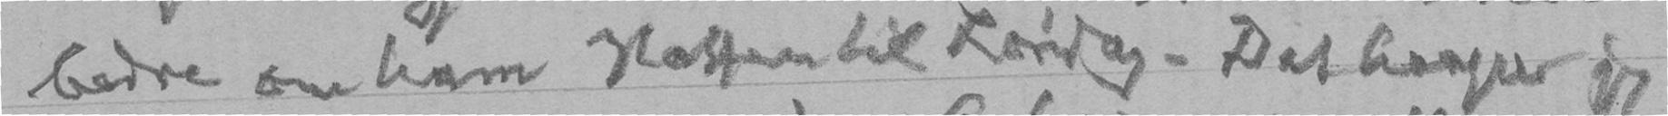bedre om ham Natten til Lørdag. Det haaper jeg
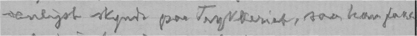venligst skynde paa Trykkeriet, saa han faar
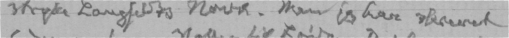stryke Langfeldts Navn. Men jeg har skrevet
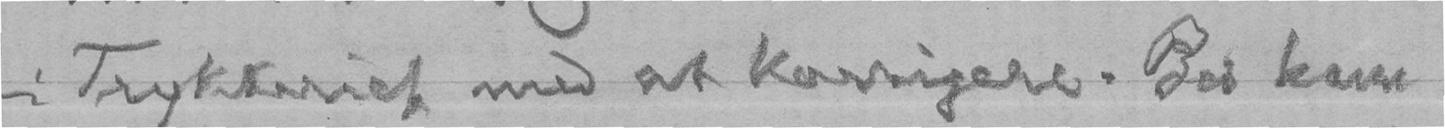i Trykkeriet med at korrigere. Bed ham
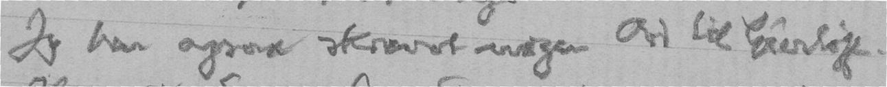Jeg har ogsaa skrevet nogen Ord til Gierløff.
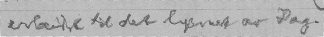arbeidet til det lysnet av Dag.
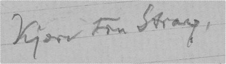Kjære Fru Stray,
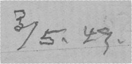3/5. 49.
